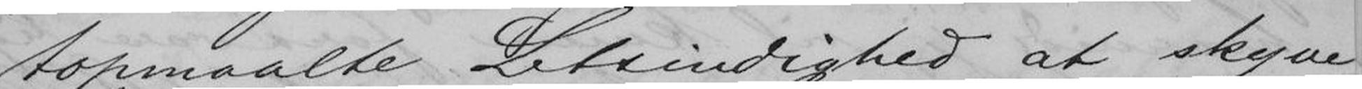topmaalte Letsindighed at skyve
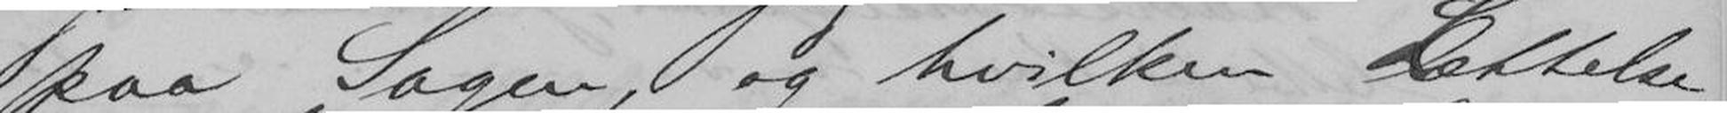paa Sagen, og hvilken Lettelse
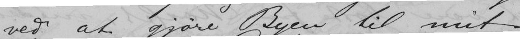ved at gjøre Byen til mit
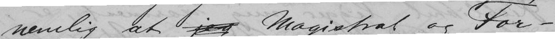nemlig at jeg Magistrat og For-
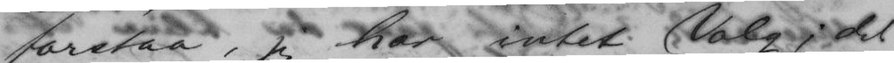forstaa, jeg har intet Valg, det
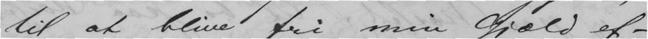til at blive fri min Gjæld ef-
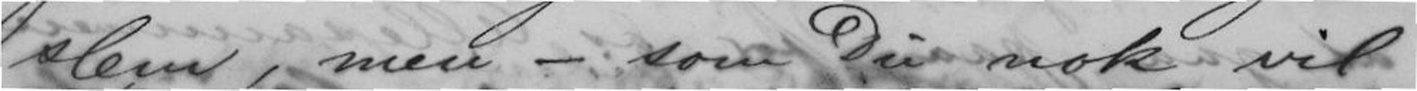slem, men - som Du nok vil
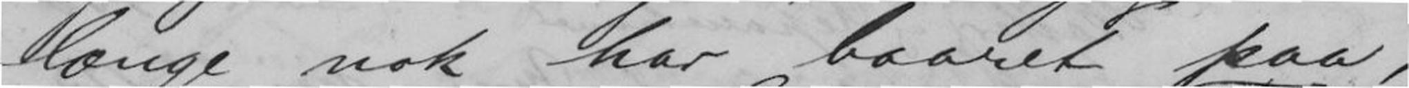længe nok har baaret paa,
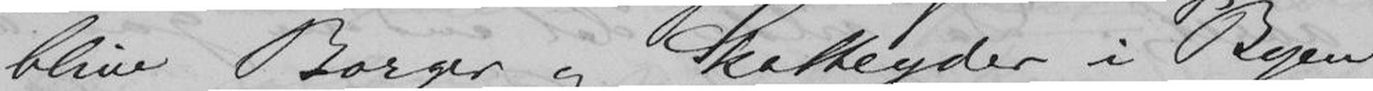blive Borger og Skatteyder i Bergen
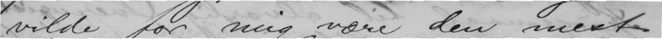vilde for mig være den mest
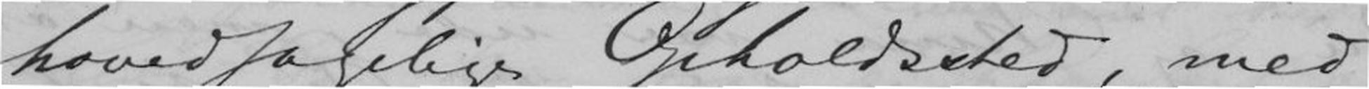hovedsagelige Opholdsted, med
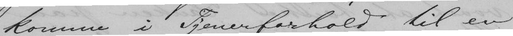komme i Tjenerforhold til en
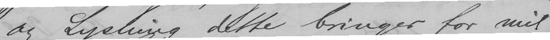og Lysning dette bringer for mit
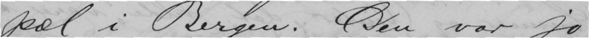pæl i Bergen. Den var jo
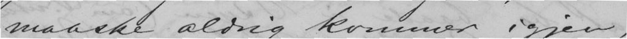maaske aldrig kommer igjen,
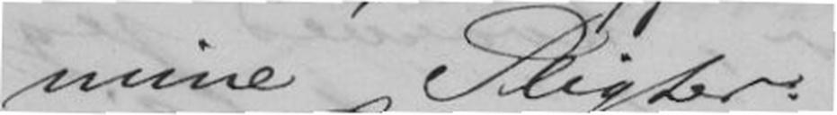mine Pligter.
